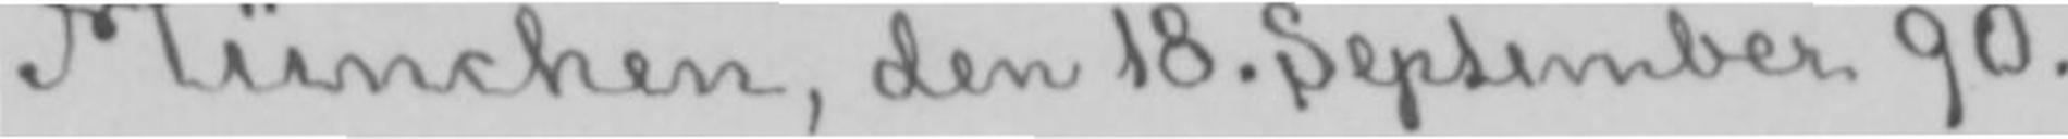München, den 18. September 90.
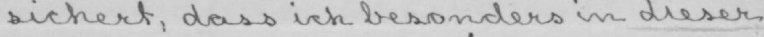sichert, dass ich besonders in dieser
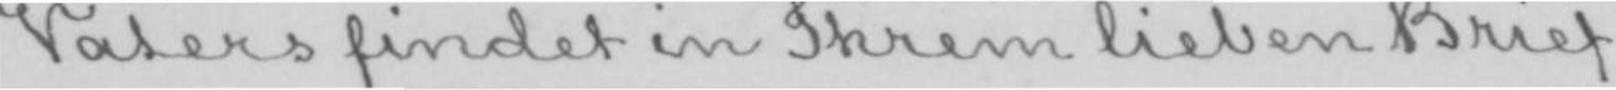Vaters findet in Ihrem lieben Brief
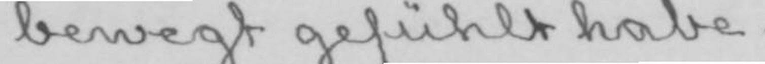bewegt gefühlt habe.
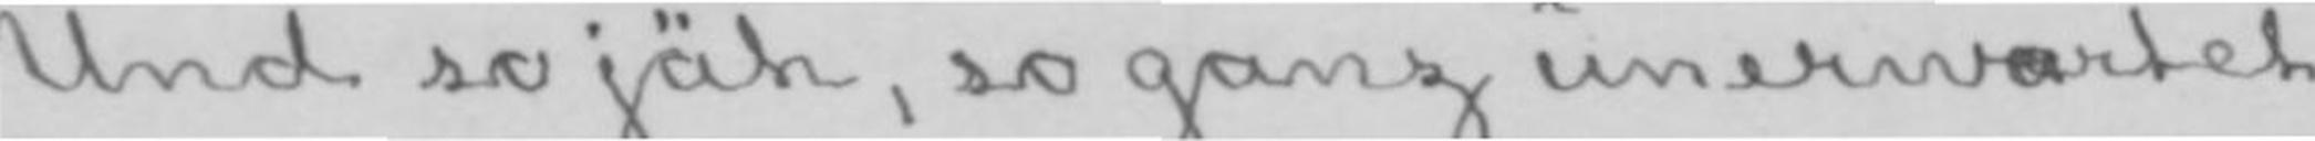Und so jäh, so ganz unerwartet
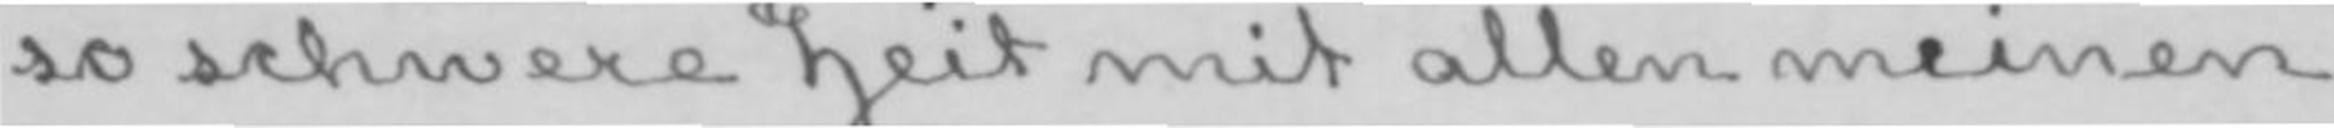so schwere Zeit mit allen meinen
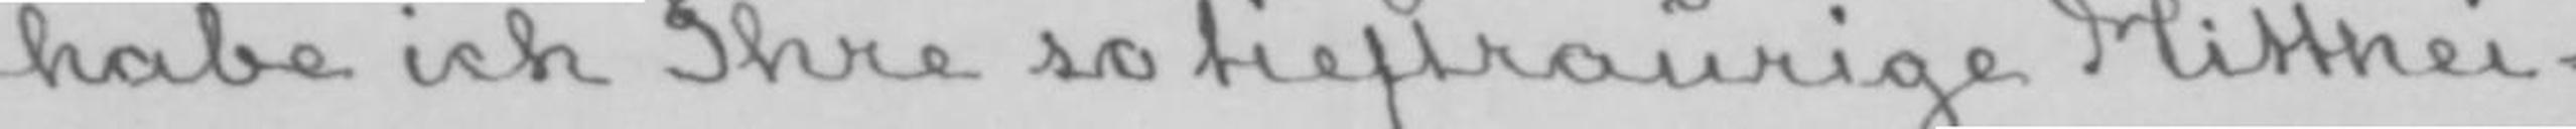habe ich Ihre so tieftraurige Mitthei-
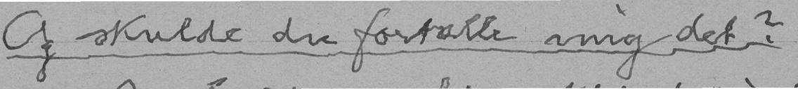Og skulde du fortælle mig det?
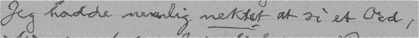Jeg hadde nemlig nektet at si et Ord,
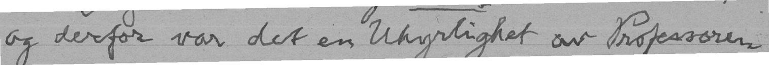og derfor var det en Uhyrlighet av Professoren
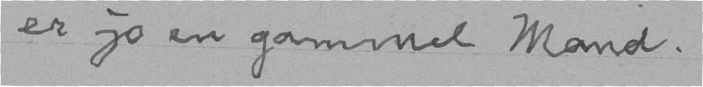er jo en gammel Mand.
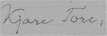Kjære Tore,
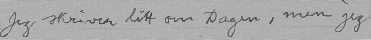Jeg skriver litt om Dagen, men jeg
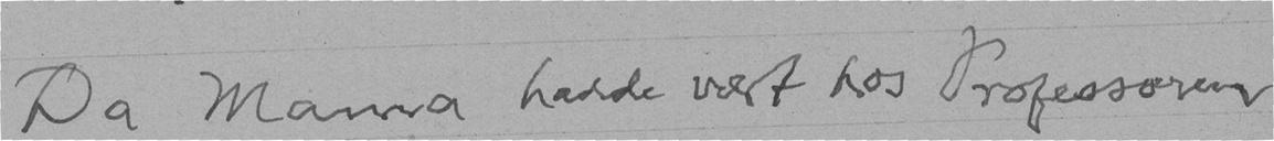Da Mama hadde vært hos Professoren
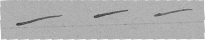- - -
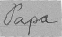Papa
